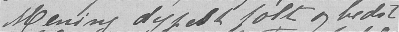Mening dypest følt og bedst
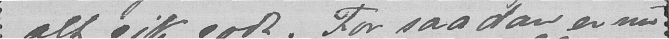alt gik godt. For saadan er nu
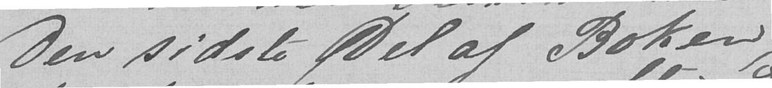Den sidste Del af Boken,
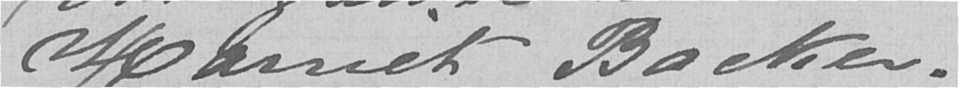Harriet Backer.
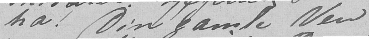ha! Din gamle Ven
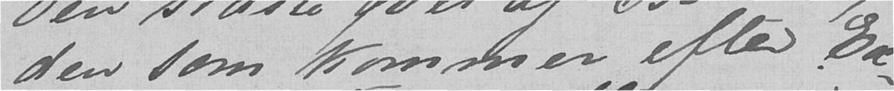den som kommer efter Ex-
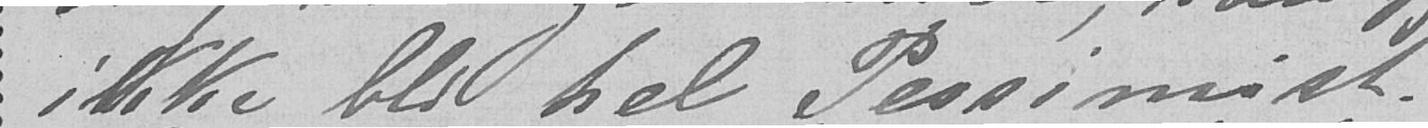ikke bli hel Pessimist.
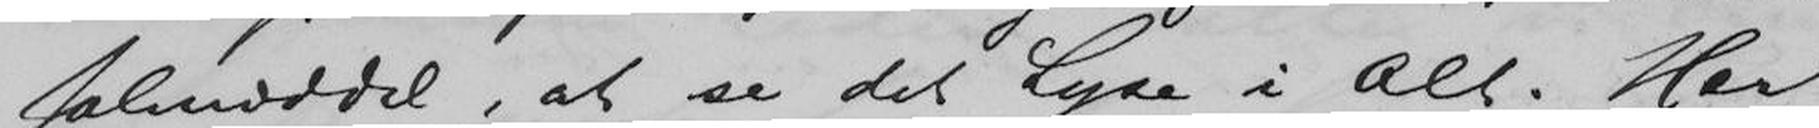salmiddel, at se det Lyse i Alt. Her
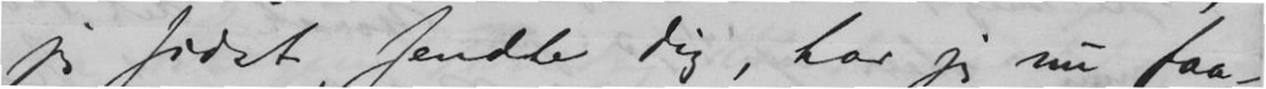jeg sidst sendte Dig, har jeg nu faa-
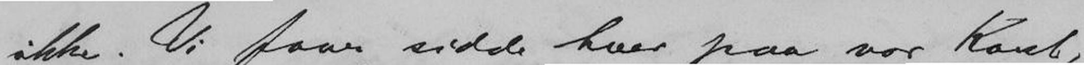ikke. Vi faar sidde hver paa vor Kant,
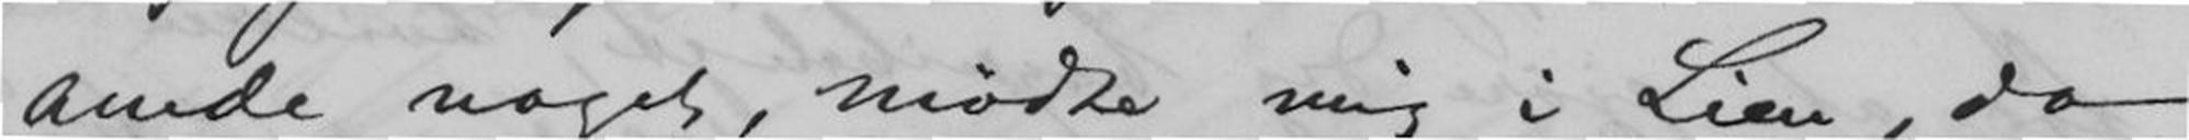anede noget, mødte mig i Lien, da
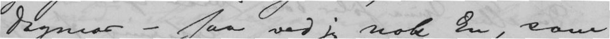Dagmar - saa ved jeg nok En, som
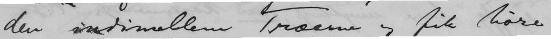den indimellem Træerne og fik høre
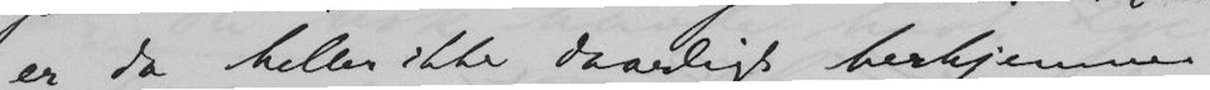er da heller ikke daarligt herhjemme,
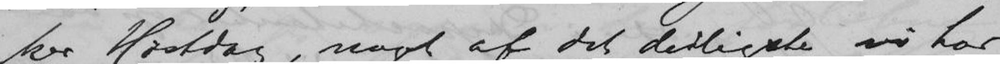ker Høstdag, noget af det deiligste vi har
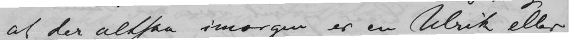at der altsaa imorgen er en Ulrik eller
